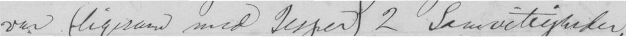var (ligesom med Jesper) 2 Samvitigheder,
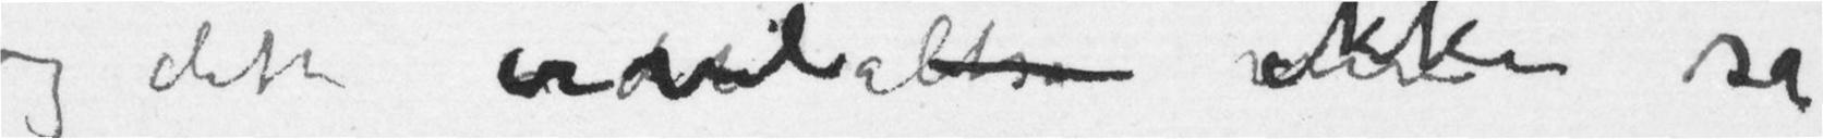og dette er vel altså ikke så
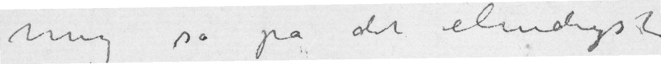mig så på det elendigste
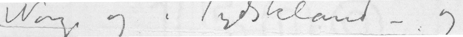Norge og Tydskland – og
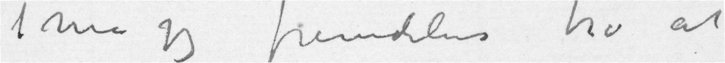må jeg fremdeles tro at
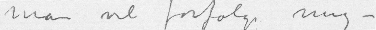man vil forfølge mig –
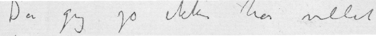Da jeg jo ikke har villet
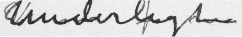Underligt
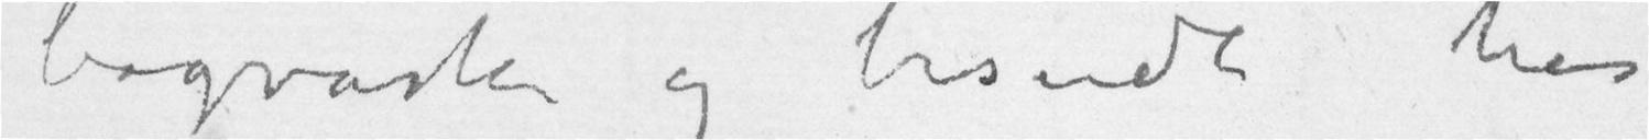bagvaske og besudle har
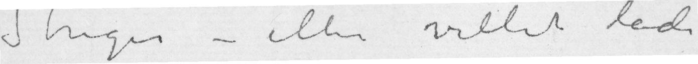Streger – eller villet lade
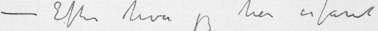– Efter hvad jeg har erfaret
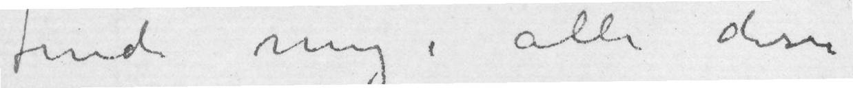finde mig i alle disse
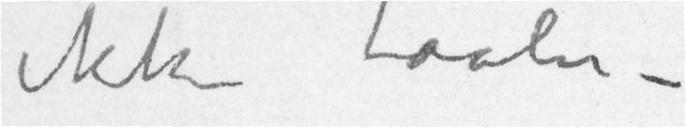ikke taaler –
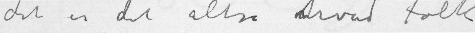det er altså hvad Folk
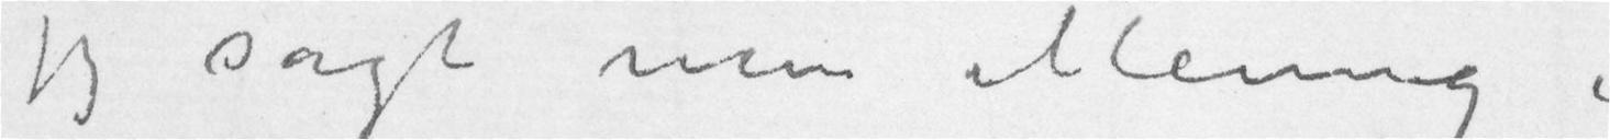jeg sagt min Mening i
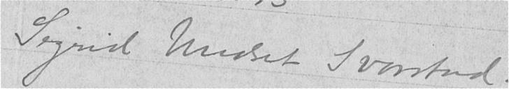Sigrid Undset Svarstad.
